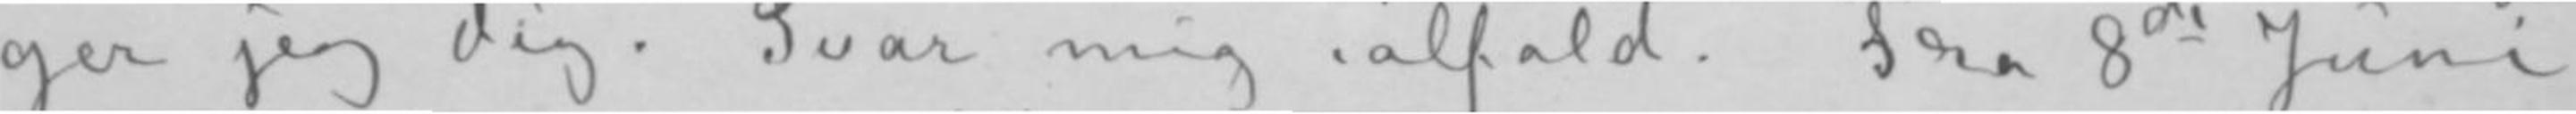ger jeg Dig. Svar mig ialfald. Fra 8de Juni
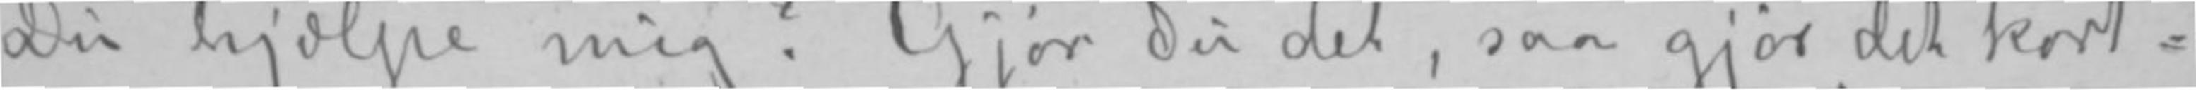Du hjælpe mig? Gjør Du det, saa gjør det kort-
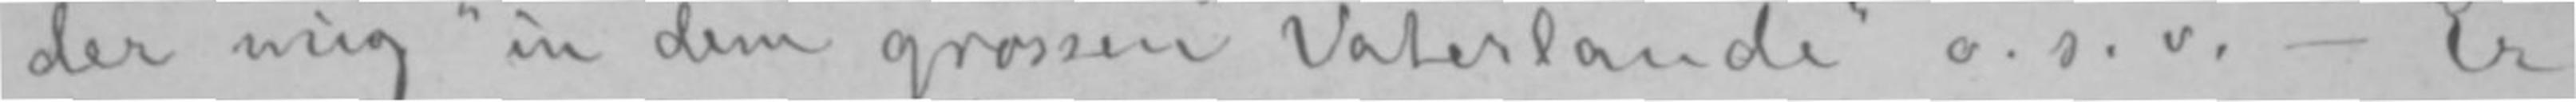der mig «in dem grossen Vaterlande» o. s. v.- Er
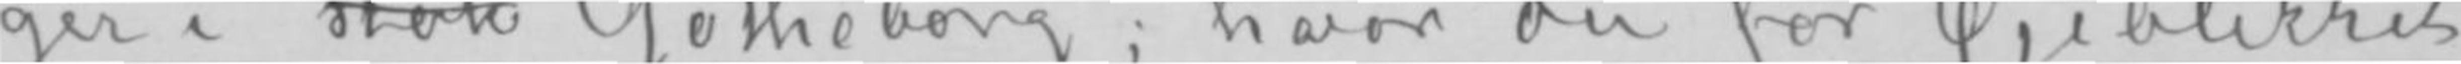ger i Stok Götheborg; hvor Du for Øjeblikket
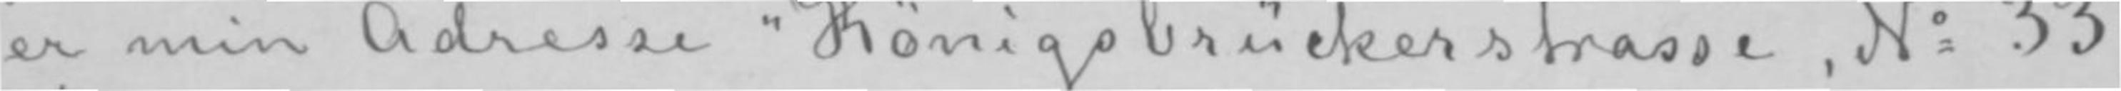er min Adresse «Königsbrückerstrasse, No 33,
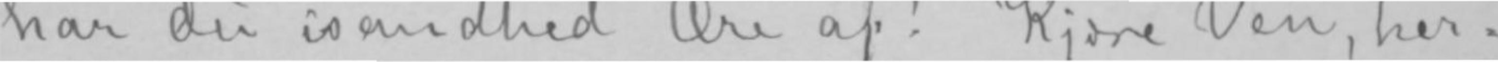har Du isandhed Ære af! Kjære Ven, her-
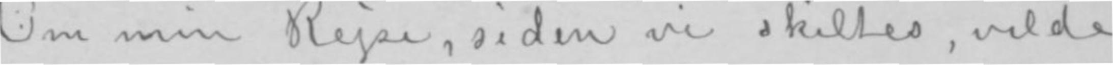Om min Rejse, siden vi skiltes, vilde
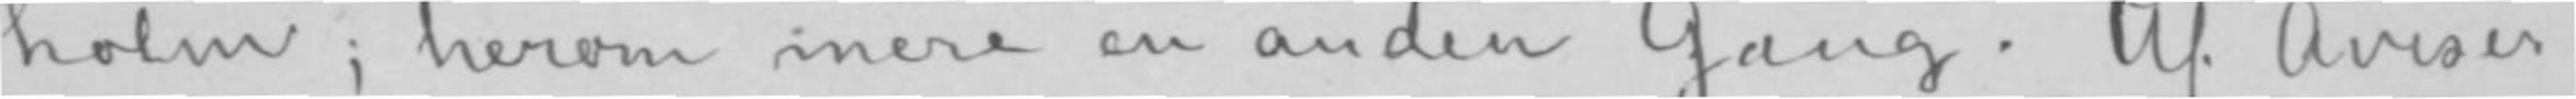holm; herom mere en anden Gang. Af Aviser-
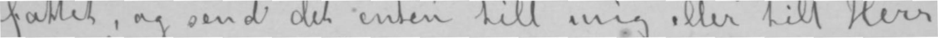fattet, og send det enten till mig eller till Herr
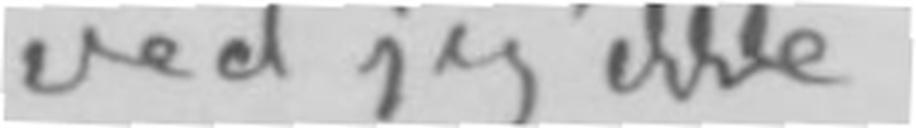ved jeg ikke
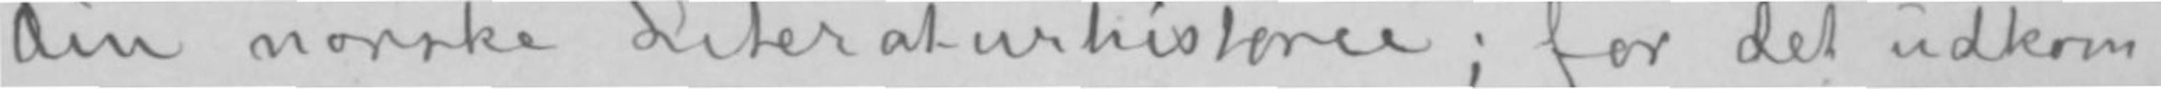Din norske Literaturhistorie; for det udkom-
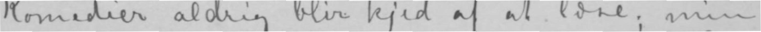Komedier aldrig blir kjed af at læse; min
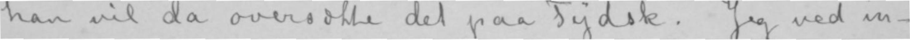han vil da oversætte det paa Tydsk. Jeg ved in-
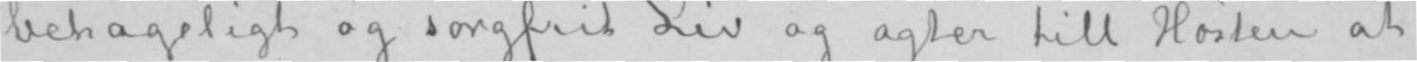behageligt og sorgfrit Liv og agter till Høsten at
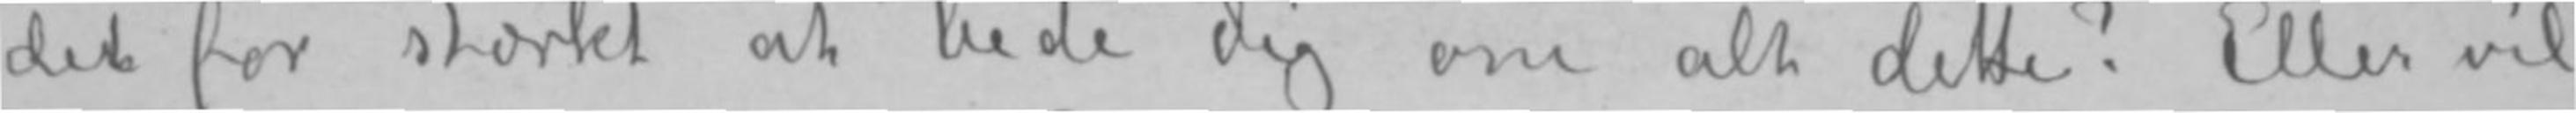det for stærkt at bede Dig om alt dette? Eller vil
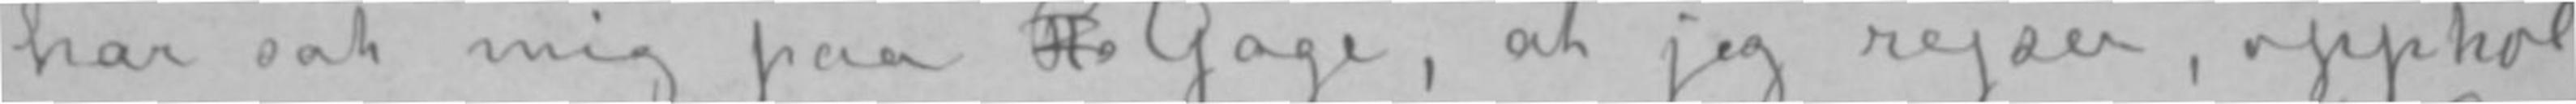har sat mig paa Ro Gage, at jeg rejser, opphol-
